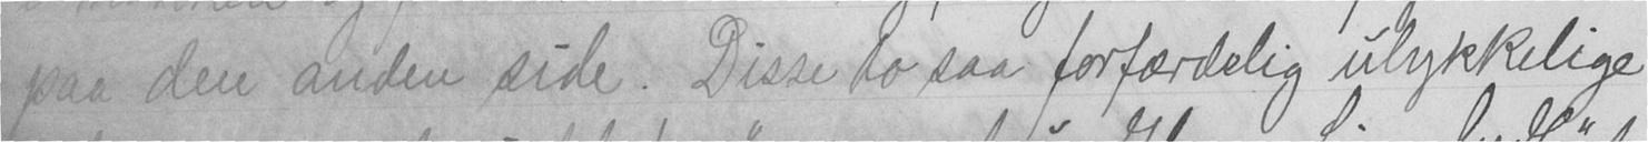paa den anden side. Disse to saa forfærdelig ulykkelige
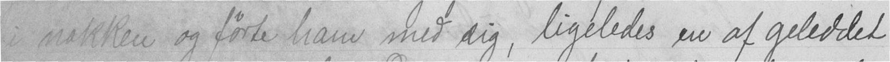i nakken og førte ham med sig, ligeledes en af geleddet
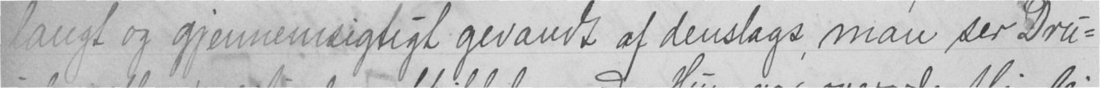langt og gjennemsigtigt gevandt af denslags, man ser Dru-
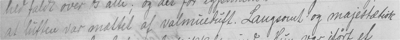at luften var mættet af valmueduft. Langsomt og majestætisk
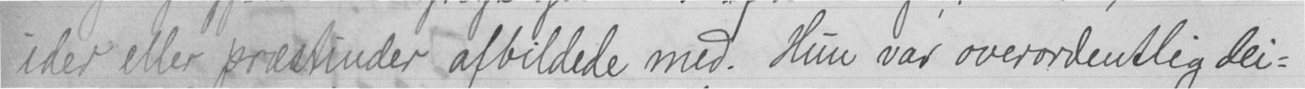ider eller præstinder afbildede med. Hun var overordentlig dei-
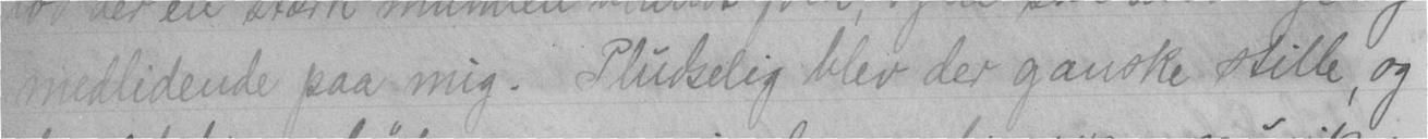medlidende paa mig. Pludselig blev der ganske stille, og
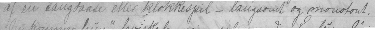af en sangdaase eller klokkespil - langsomt og monotont.
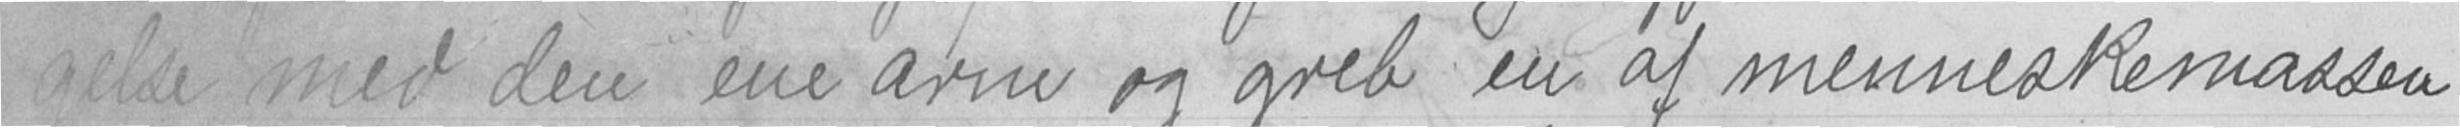gelse med den ene arm og greb en af menneskemassen
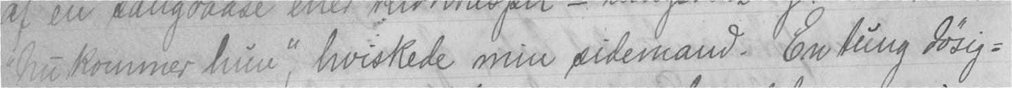"Nu kommer hun", hviskede min sidemand. En tung døsig-
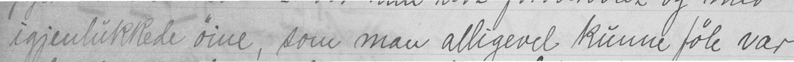igjenlukkede øine, som man alligevel kunne føle var
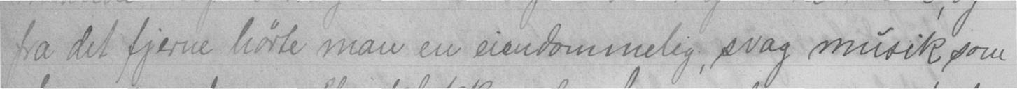fra det fjerne hørte man en eiendommelig, svag musik som
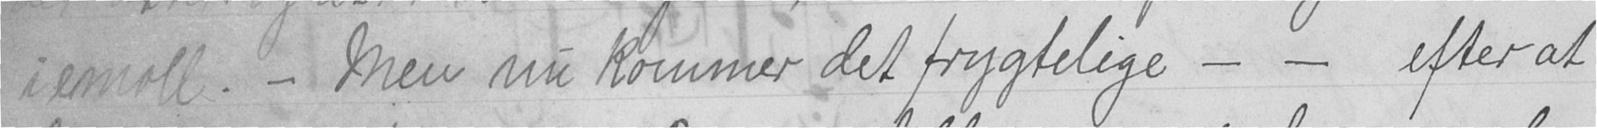i emoll. - Men nu kommer det frygtelige - - efter at
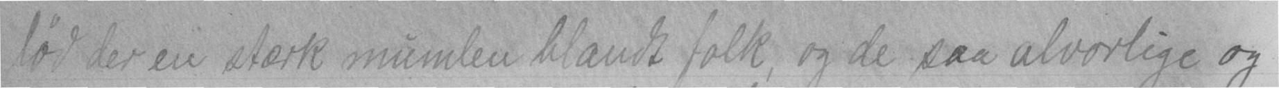lød der en stærk mumlen blandt folk, og de saa alvorlige og
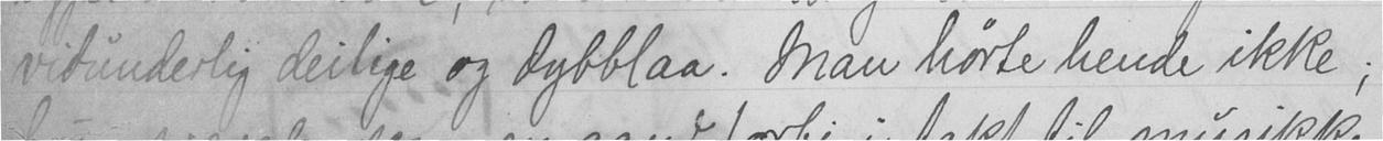vidunderlig deilige og dybblaa. Man hørte hende ikke;
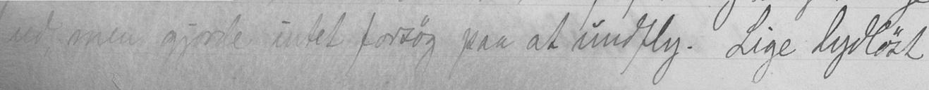ud, men gjorde intet forsøg paa at undfly. Lige lydløst
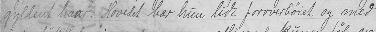gyldent haar. Hovedet bar hun lidt foroverbøiet og med
